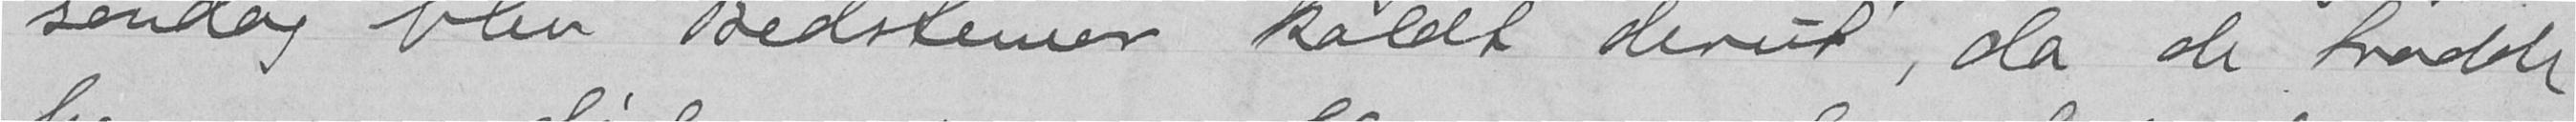søndag blev Bedstemer kaldt derut, da de trodde
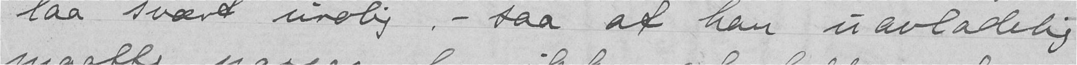laa svært urolig ,- saa at han uarladelig
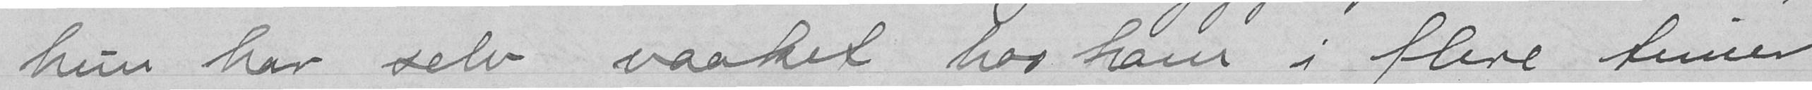hun har selv vaaket hos ham i flere timer
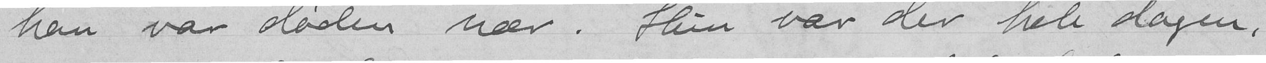han var døden hær. Hun var der hele dagen,
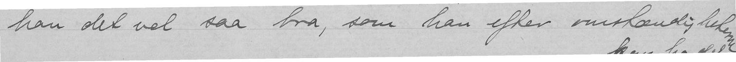han det vel saa bra, som han efter omstændighetene
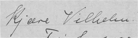Kjære Vilhelm.
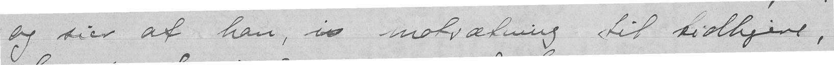og sier at han, i motsætning til tidligere
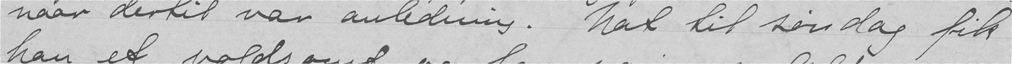naar dertil var anledning. Nat til sendag fik
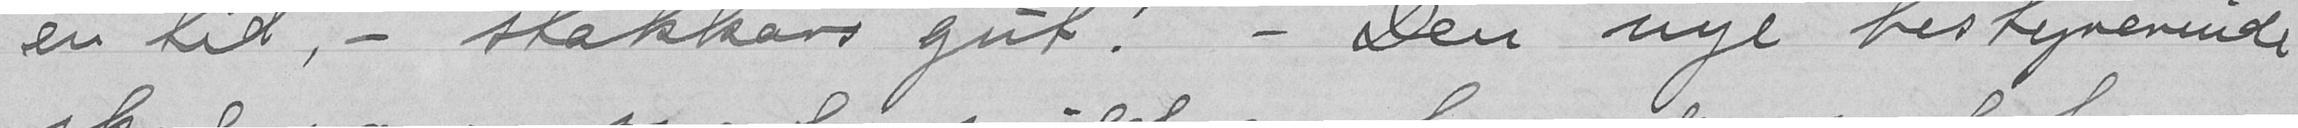en tid, - stakkars gut! - Den nye bestyrerinde
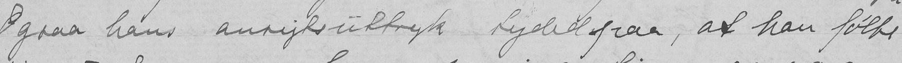Ogsaa hans ansigtsutrykk tyded paa at han følte
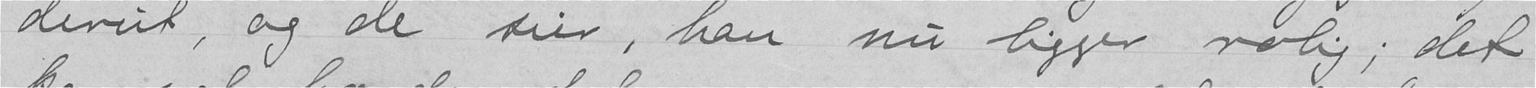derut, og de sier, han nu ligger rolig; det
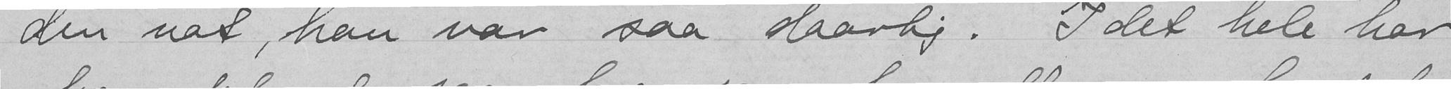den nat, han var saa daarlig. I det hele har
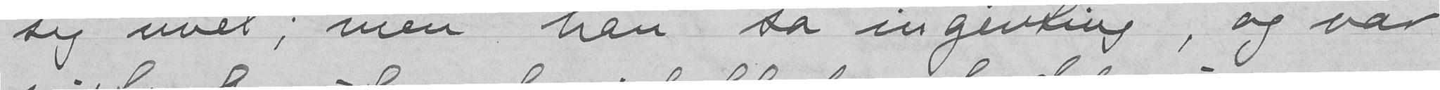sig uvel; men han sa ingenting, og var
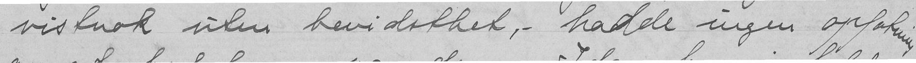vistnok ulen bevidsthet,- hadde ingen opfatning
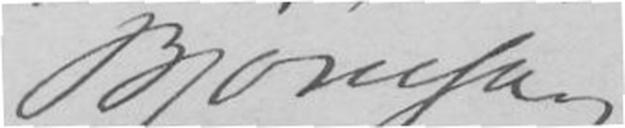Bjørnson
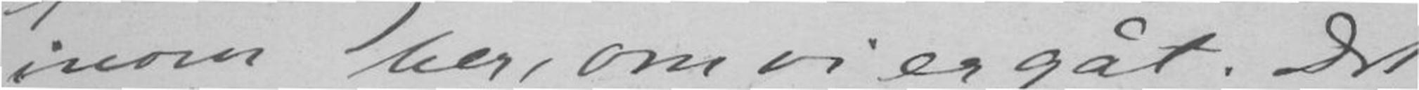inom her, om vi er gåt. Det
